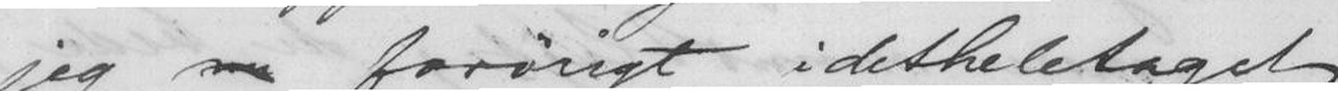jeg nu forøvrigt idetheletaget
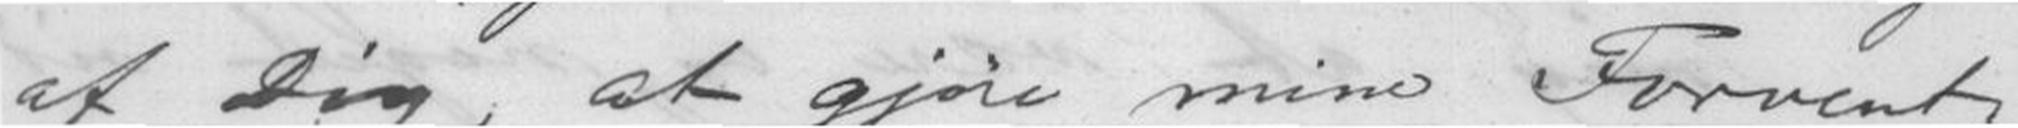af Dig, at gjøre mine Forvent-
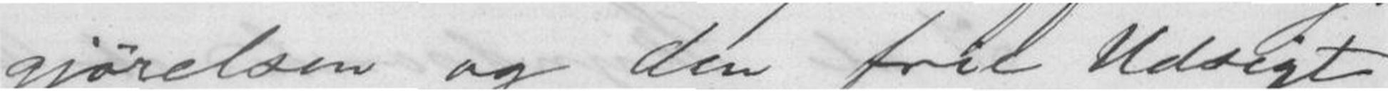gjørelsen og den frie Udsigt
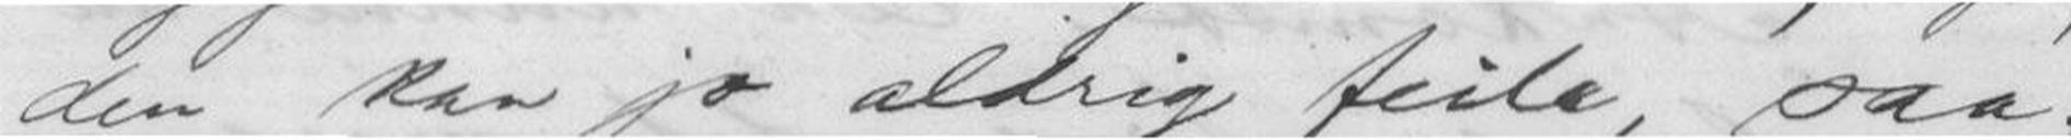den kan jo aldrig feile, saa-
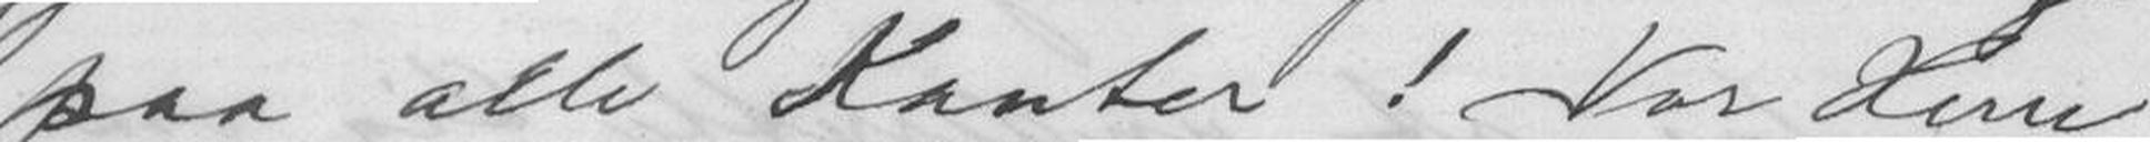paa alle Kanter! Vor Herre
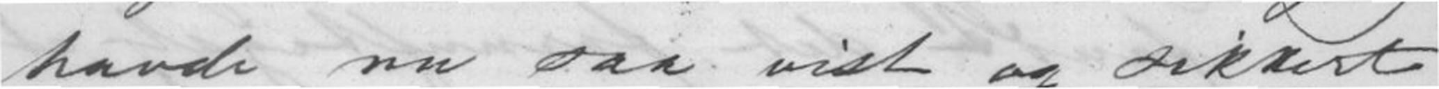havde nu saa vist og sikkert
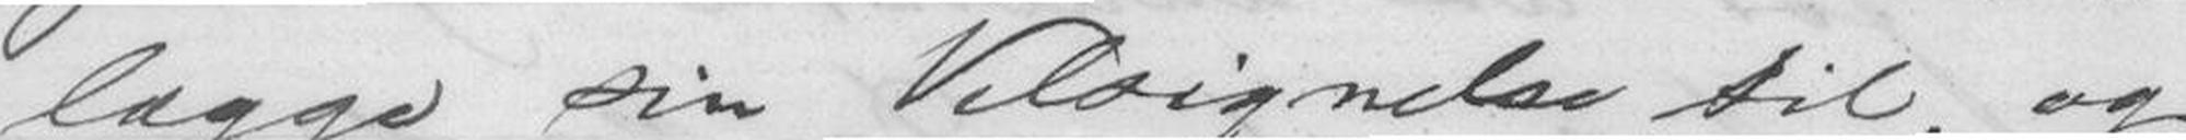lægge sin Velsignelse til, og
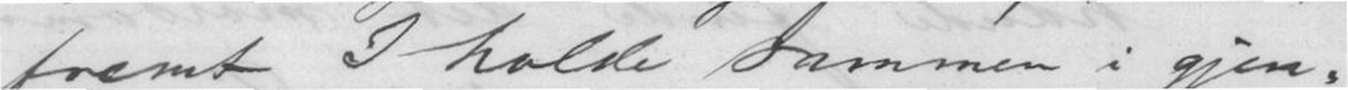fremt I holde sammen i gjen-
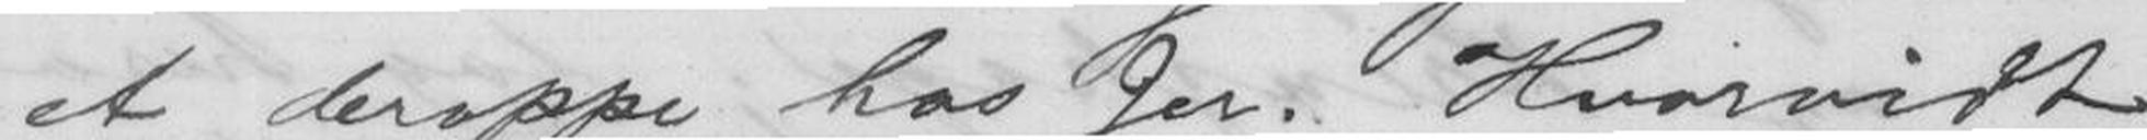et deroppe hos Jer. Hvorvidt
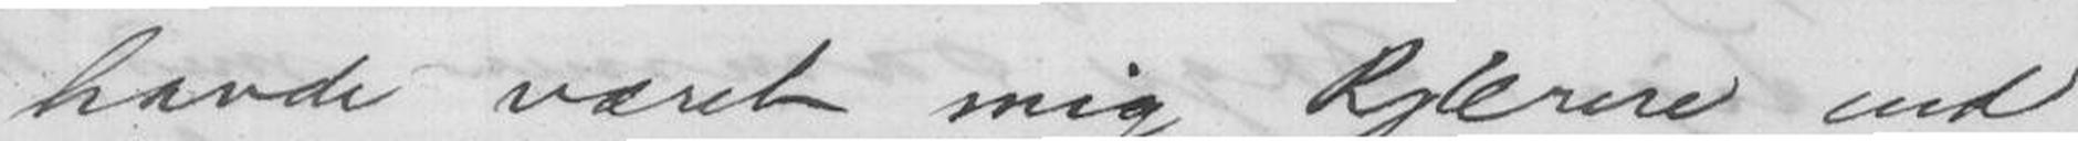havde været mig kjærere end
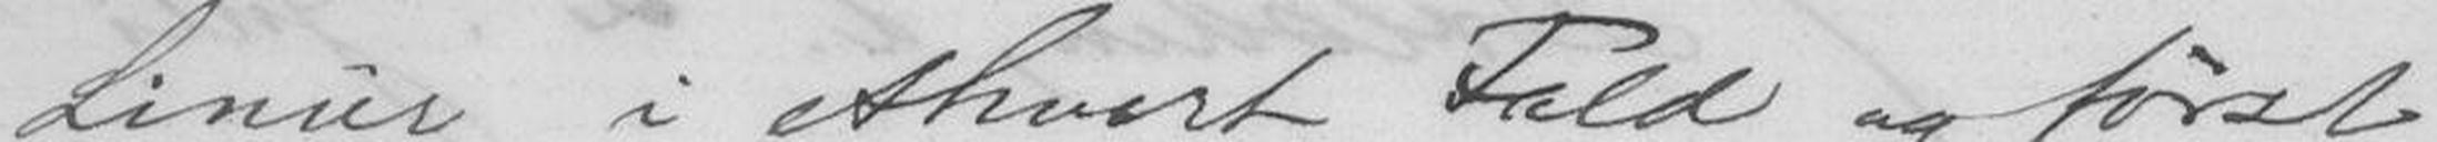Linier i ethvert Fald og først
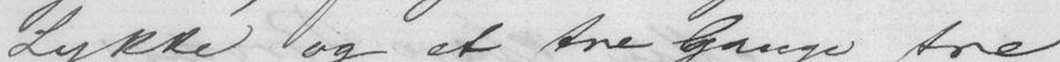Lykke og et tre Gange tre
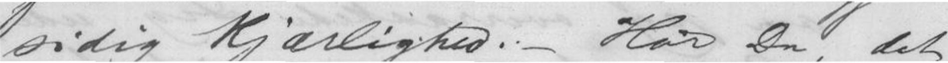sidig Kjærlighed. - Hør Du, det
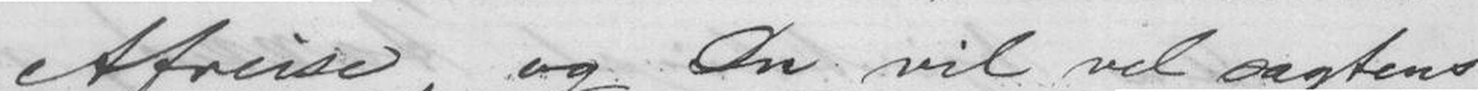Afreise, og Du vil vel sagtens
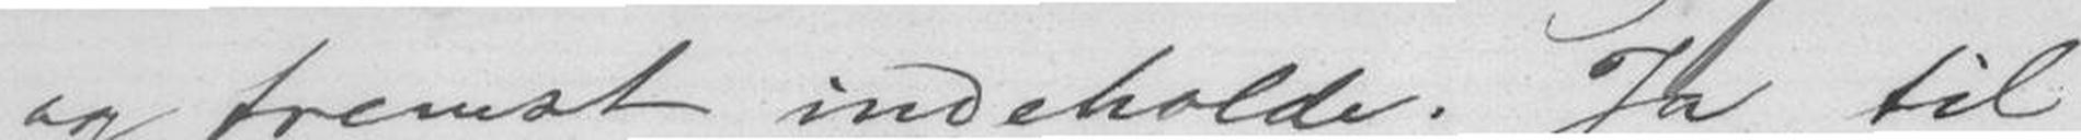og fremst indeholde. Ja, til
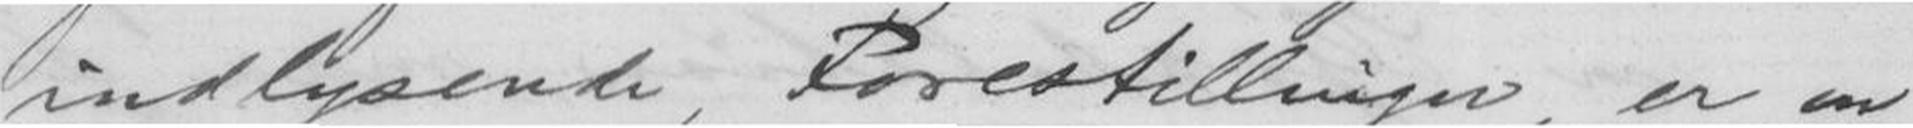indlysende, Forestillinger, er en
